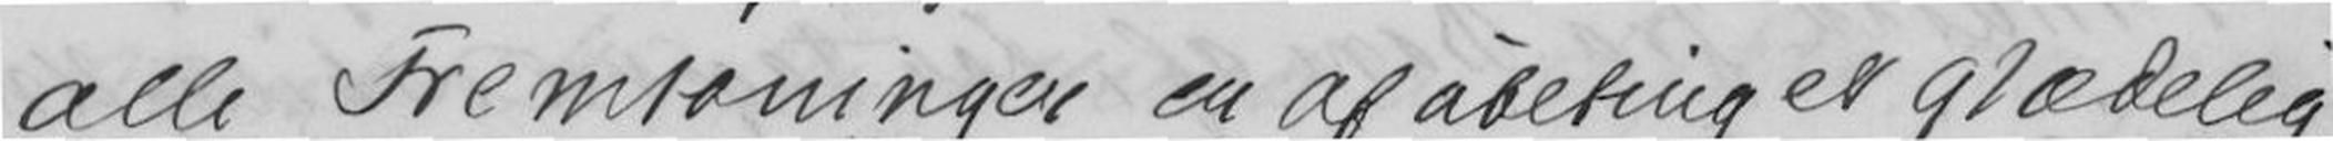alle Fremtoninger er af et glædelig
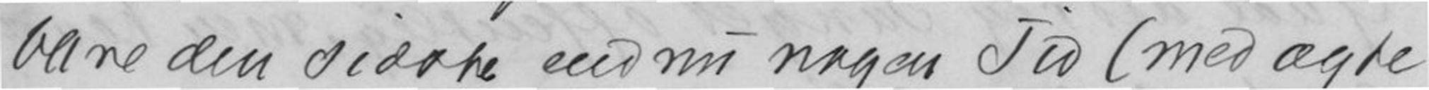bare den sidste endnu nogen Tid (med ægte
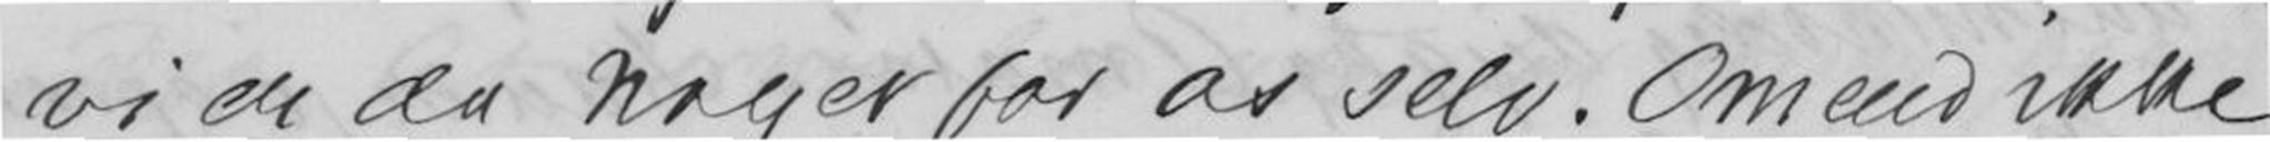vi de da noget for os selv. Om end ikke
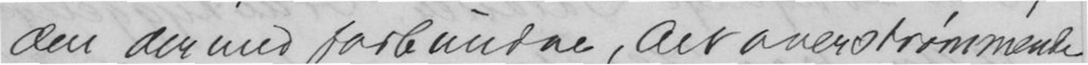den der med forbundne, Alt overstrømmende
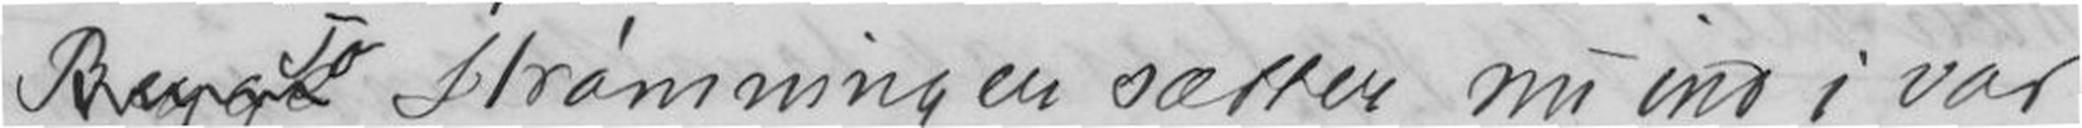overstrykn. Jo strømmingen sætter nu ind i vor
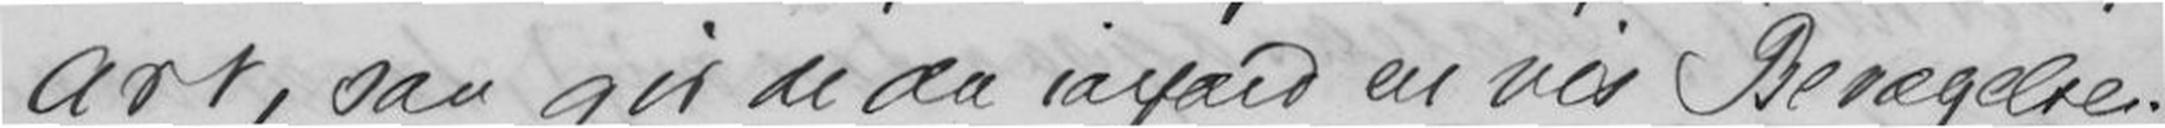art, saa gir de da ialfald en vis Bevægelse.
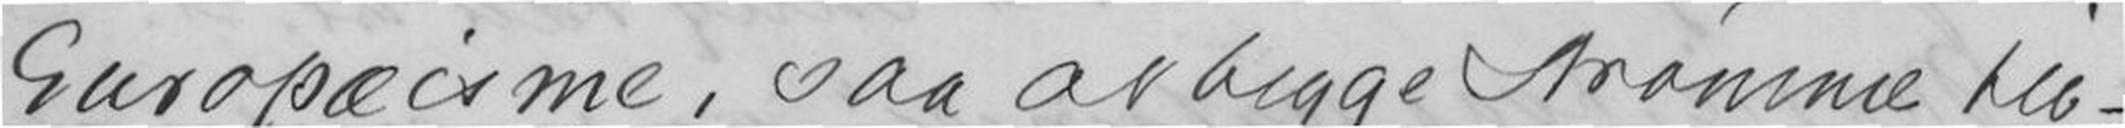Europæisme, saa at begge strømme til-
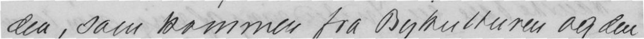den, som kommer fra Bykulturen og den
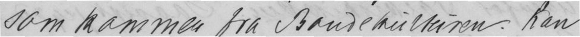som kommer fra Bondekulturen. an
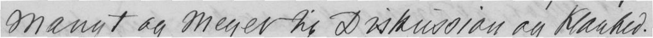mangt og meget til Diskussion og Klarhed.
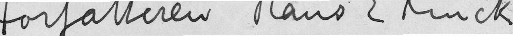Forfatteren Hans E Kinck
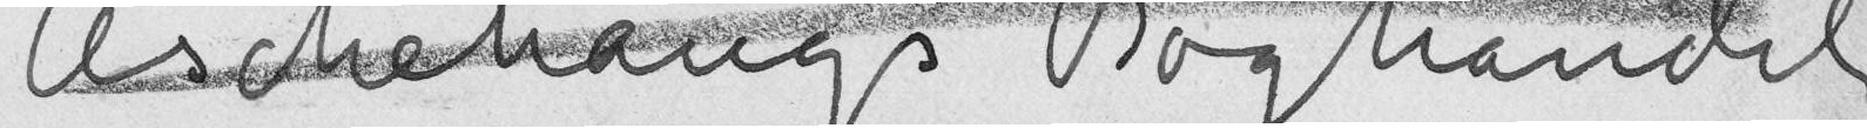Aschehougs Boghandel
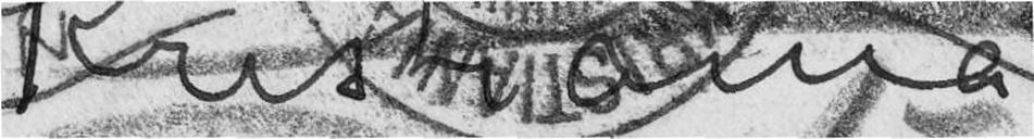Kristiania
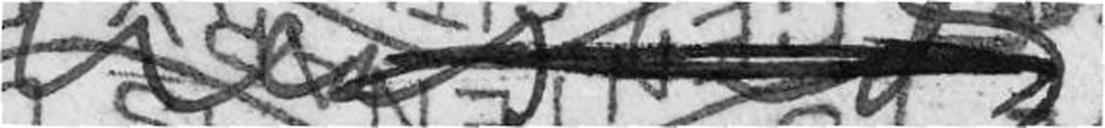Hersteds
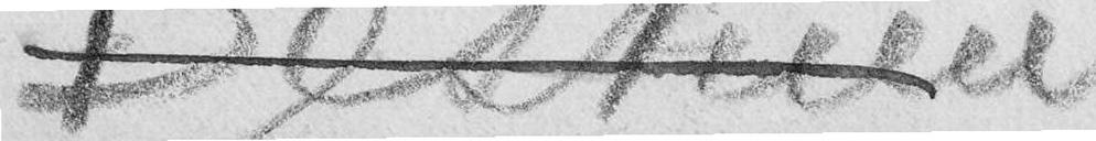Bestum
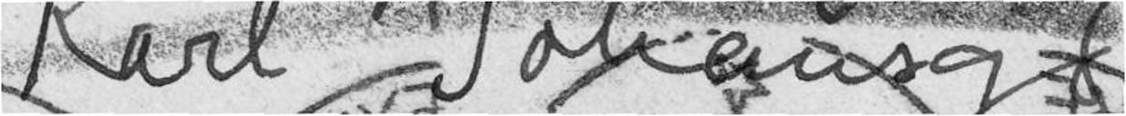Karl Johansgd
